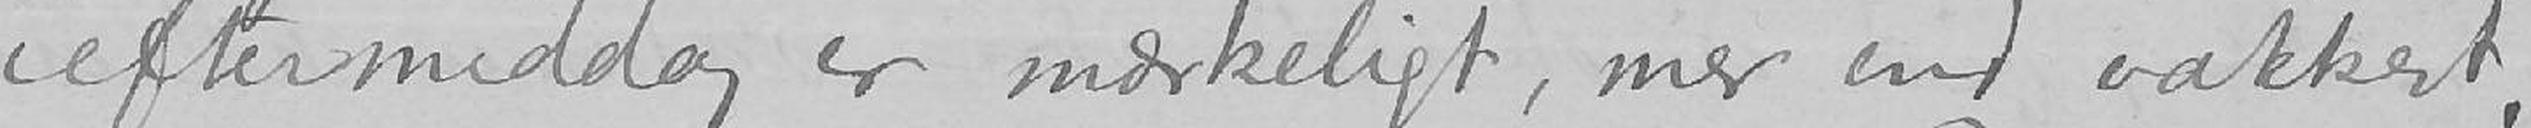ieftermiddag er mærkeligt, mer end vakkert,
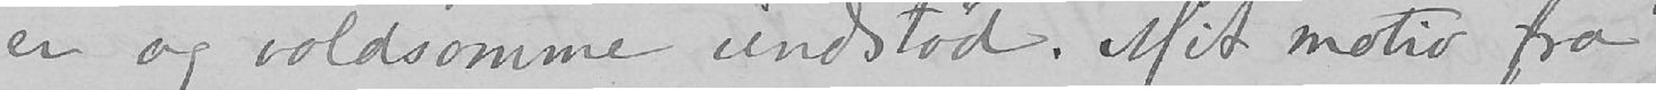er og voldsomme vindstød. Mit motiv fra
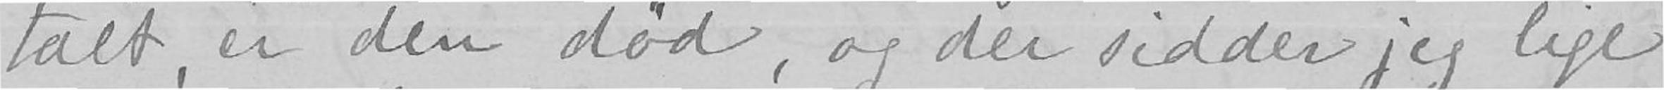talt, er den død, og der sidder jeg lige
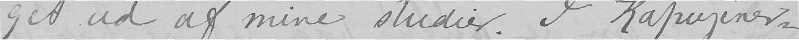get ud af mine studier. I Kapuziner-
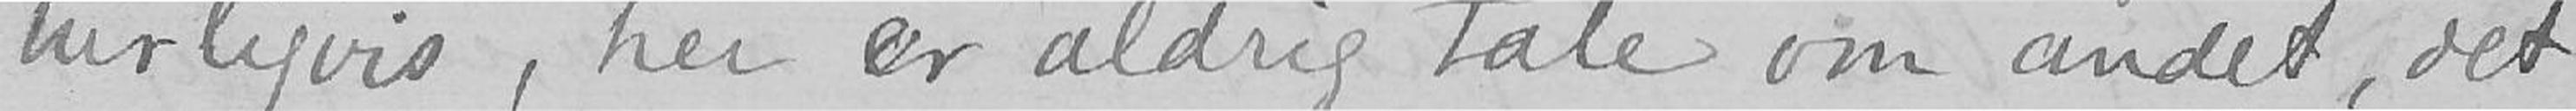turligvis, her er aldrig tale om andet, det
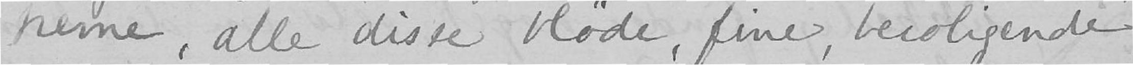perne, alle disse bløde, fine, beroligende
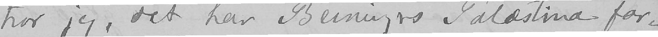tror jeg, det har Berningers Palæstina far-
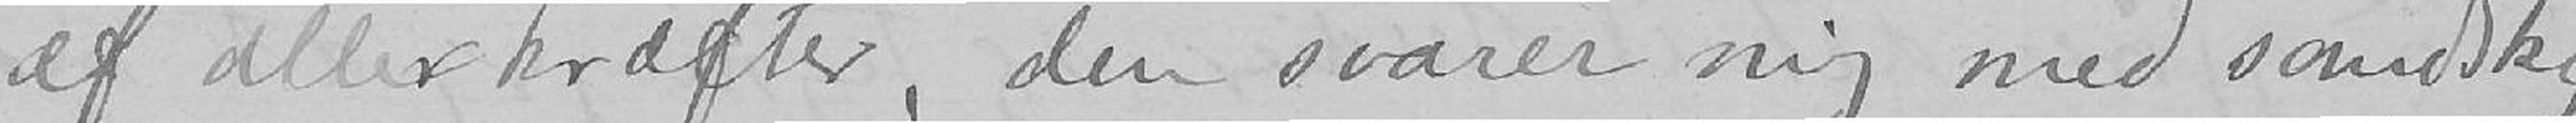af aller kræfter, den svarer mig med sandsky-
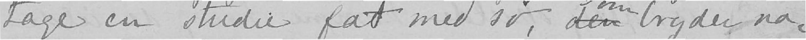tage en studie fat med sø, den som bryder na-
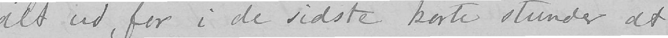alt ud, for i de sidste korte stunder at
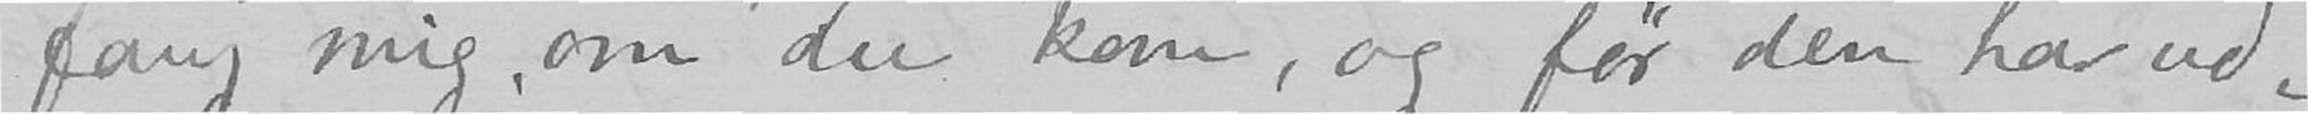fang mig, om du kan, og før den har ud-
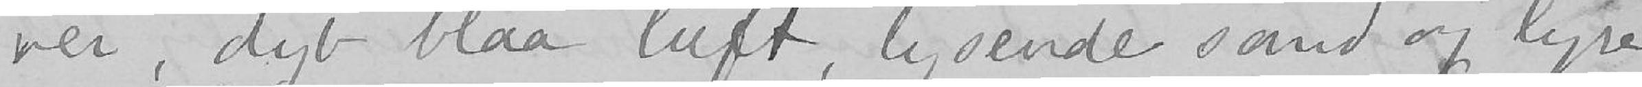ver, dyb blaa luft, lysende sand og lyse
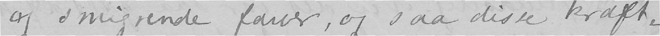og smigrende farver, og saa disse kraft-
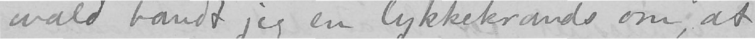wald bandt jeg en lykkekrands om, at
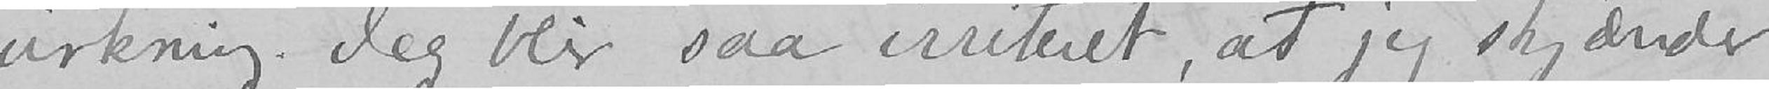virkning. Jeg blir saa irriteret, at jeg skjænder
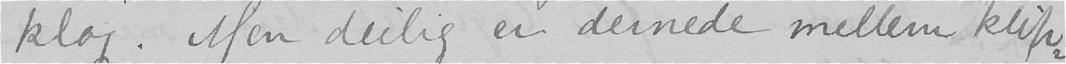klog. Men deilig er dernede mellem klip-
